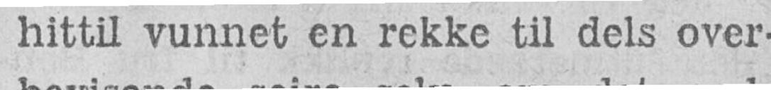hittil vunnet en rekke til dels over-
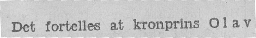Det fortelles at kronprins Olav
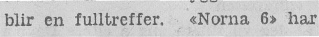blir en fulltreffer. «Norna 6» har
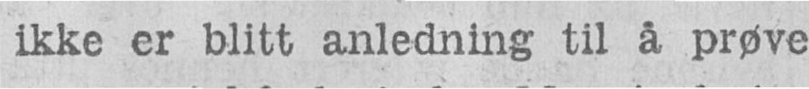ikke er blitt anledning til å prøve
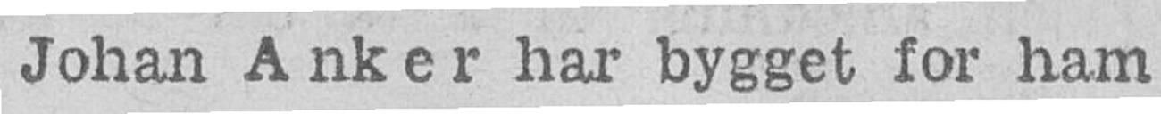Johan Anker har bygget for ham
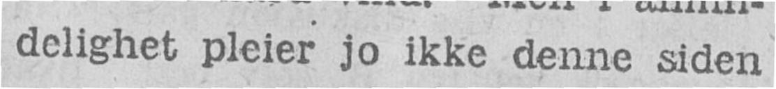delighet pleier jo ikke denne siden
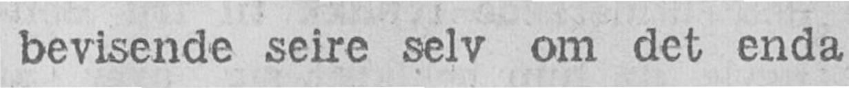bevisende seire selv om det enda
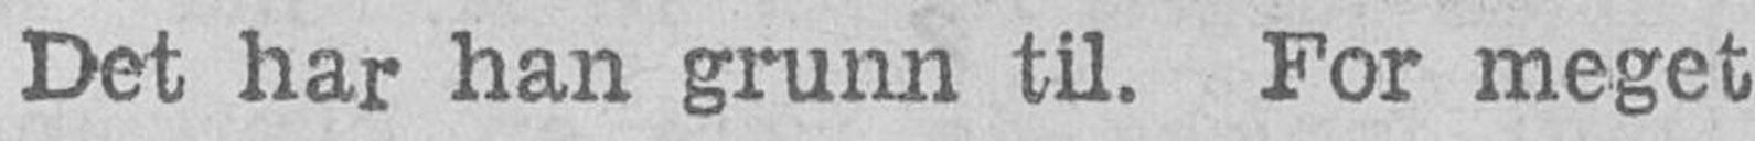Det har han grunn til. For meget
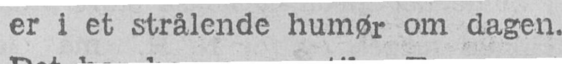er i et strålende humør om dagen.
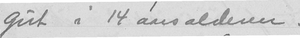gut i 14 aarsalderen.
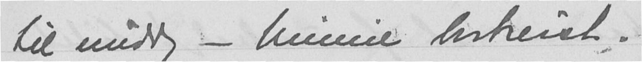til middag - Minni bortreist.
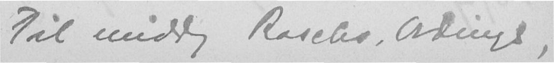Til middag Raschs, Ordings,
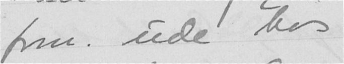form. ude hos
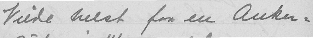Vilde helst faa en Anker-
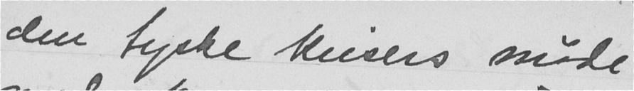den tyske keisers møde
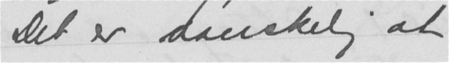Det er vanskelig at
